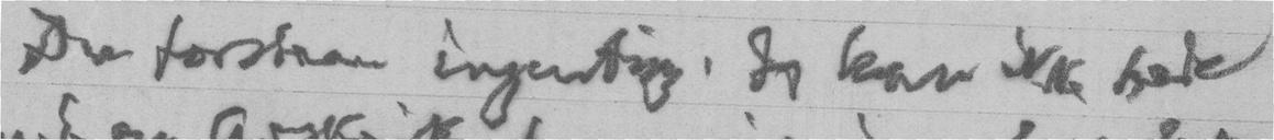Du forstaar ingenting. Jeg kan ikke bede
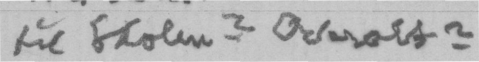til Skolen? Overalt?
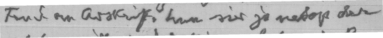Fru S. om Avskrift, hun sier jo netop der
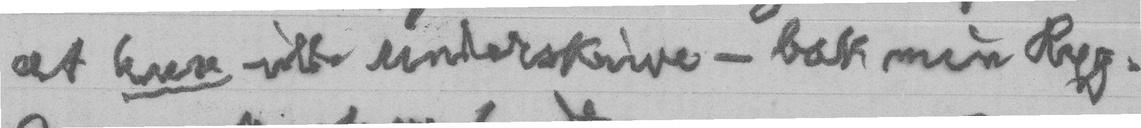at hun ville underskrive - bak min Ryg.
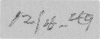12/4. 49
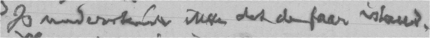Jeg underskriver ikke det de faar istand.
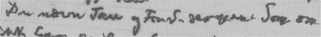Du nævn Tau og Fru S. særveere Som om
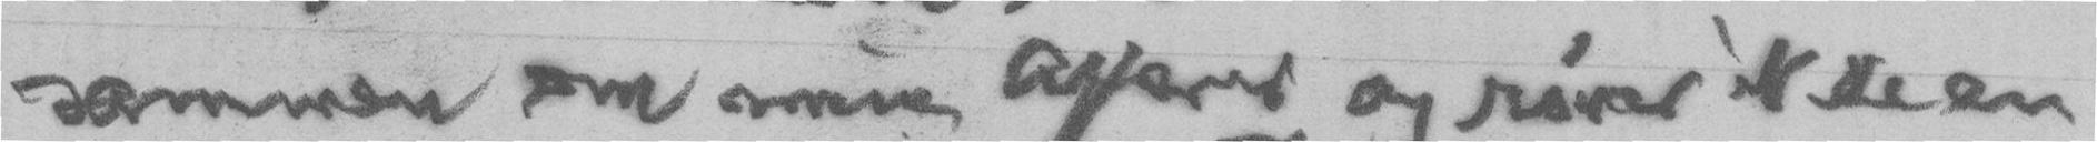sammen om mine Affærer og rører ikke en
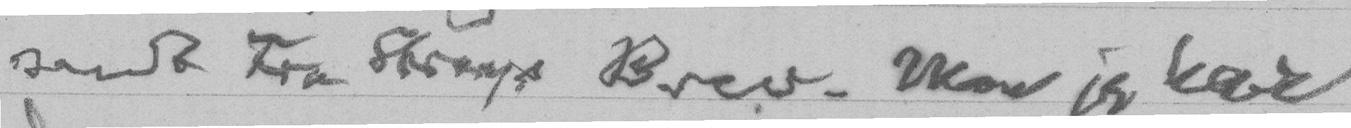sendt Fru Strays Brev. Men jeg har
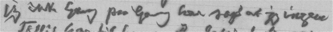jeg ikke Gang paa Gang har sagt at jeg ingen
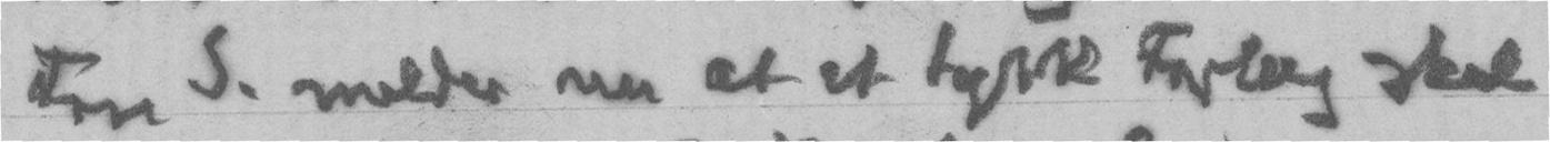Fru S. melder nu at et tysk Forlag skal
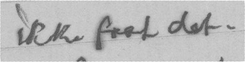ikke faat det.
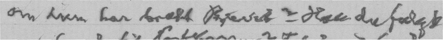om hun har brakt Brevet? Har du fulgt
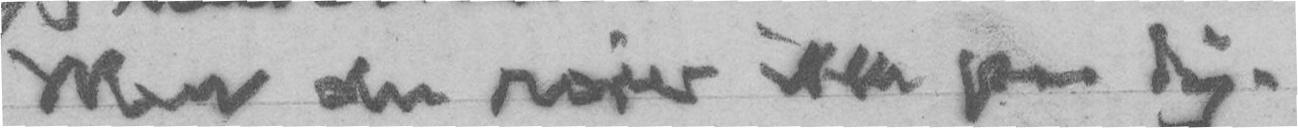Men du rører ikke paa dig.
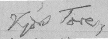Kjære Tore,
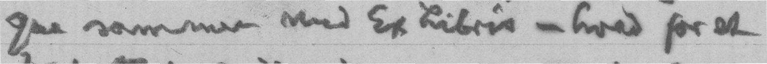gaa sammen med Ex Libris - hvad for et
